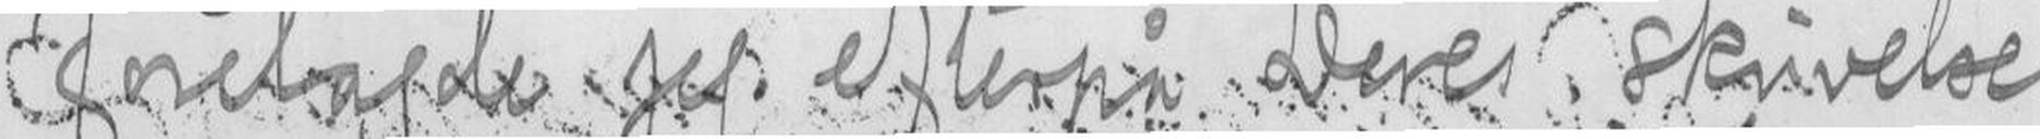foretagde jeg efterpå Deres skrivelse
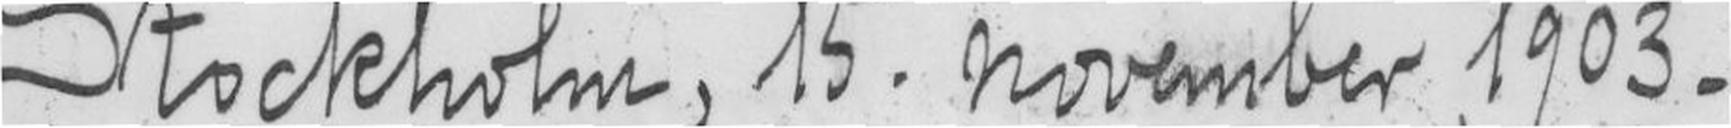Stockholm, 15 november 1903.
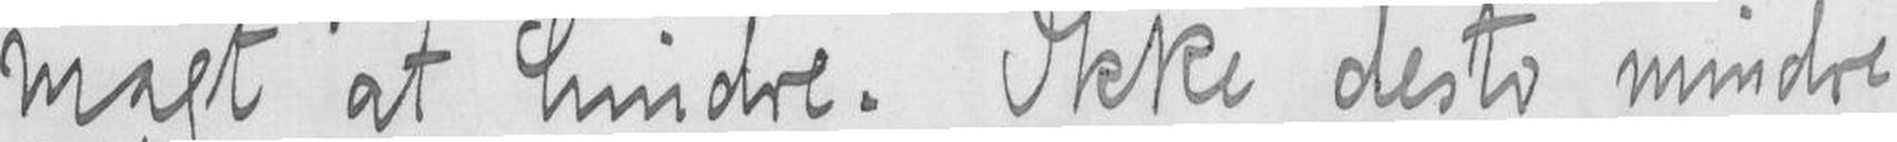magt at hindre. Ikke desto mindre
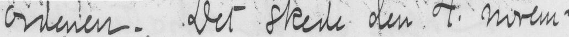ordenen. Det skede den 4 novem-
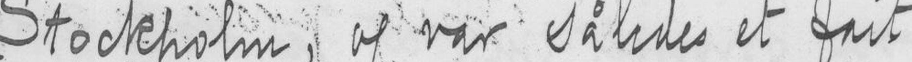Stockholm, og var således et frit
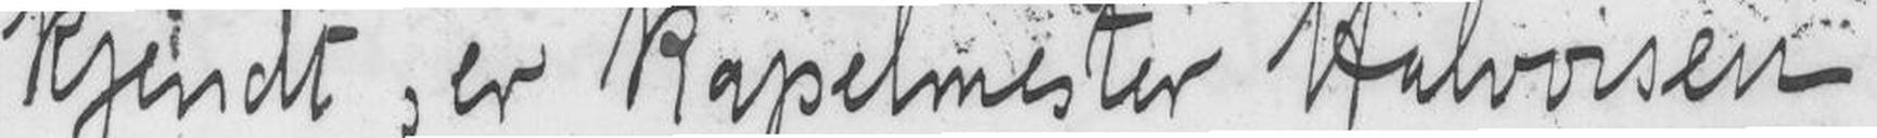kjendt, er kapelmester Halvorsen
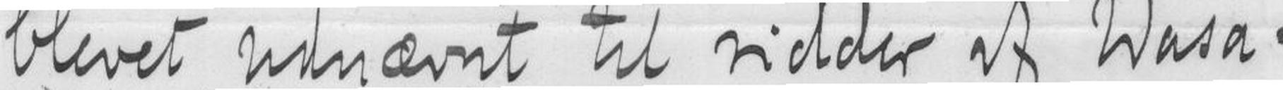blevet udnævnt til ridder af Wasa-
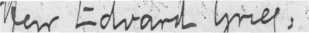Herr Edvard Grieg,
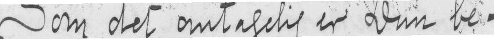Som det antagelig er Dem be-
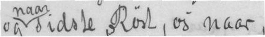og sidste Røst, os naar,
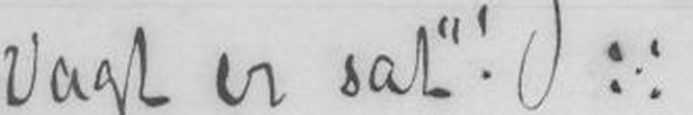Vagt et sat! :/:
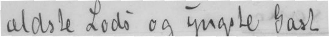ældste Lods og yngste Gast
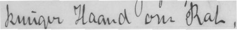knuger Haand om Rat.
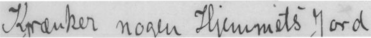Krænker nogen Hjemmets Jord,
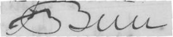JBBull
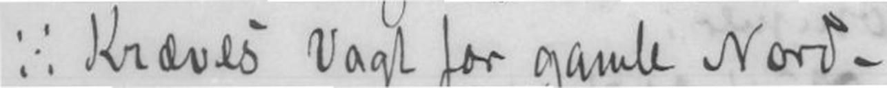:/: Kræves Vagt for gamle Nord-
